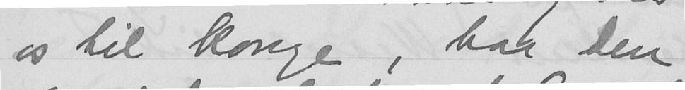os til konge, har den
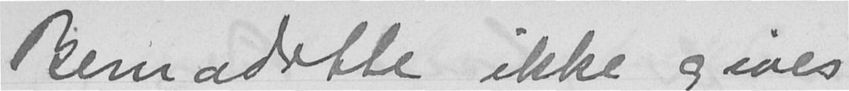Bernadotte ikke gives
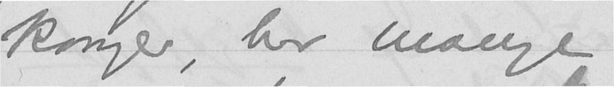konger, har mange
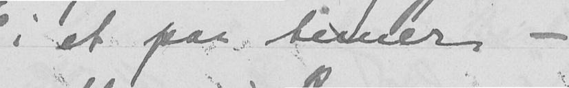i et par timer -
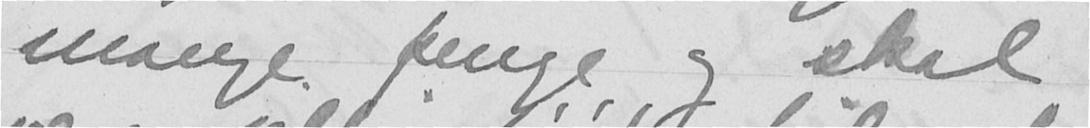mange penge og skal
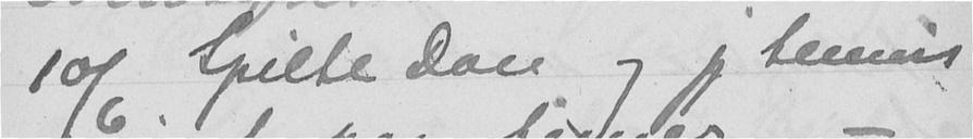10/6 Spilte Don og jeg tennis
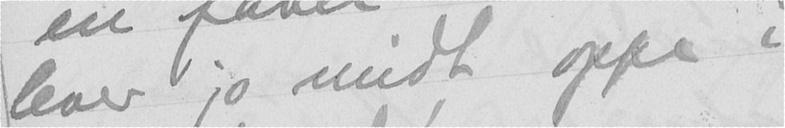boer jo midt oppe i
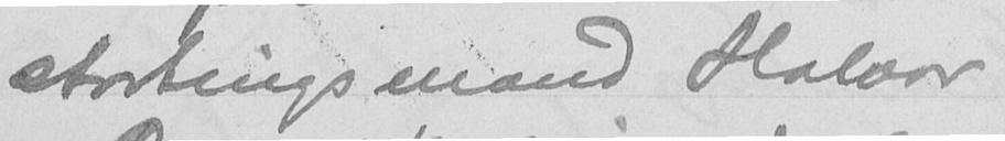stortingsmand Halvor
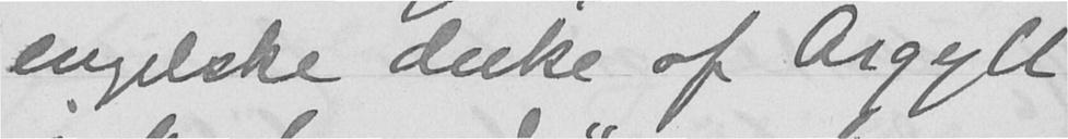engelske duke of Argyll
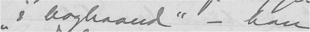"i baghaand" - han
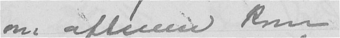om aftenen kom
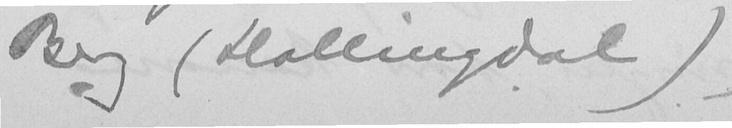Berg (Hallingdal)
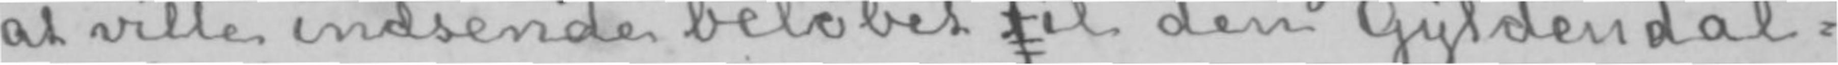at ville indsende beløbet ftil den Gyldendal-
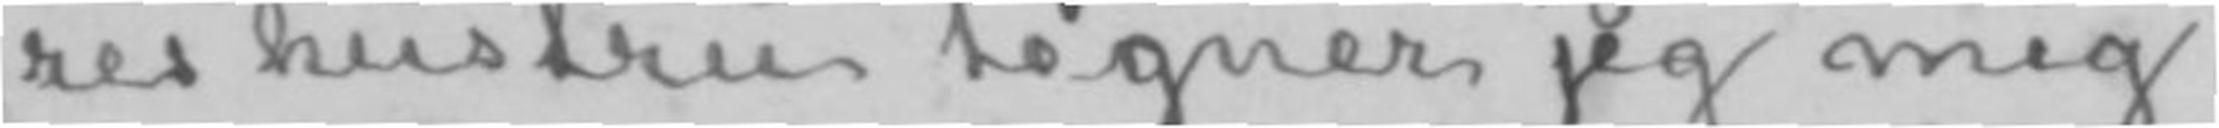res hustru tegner jeg mig
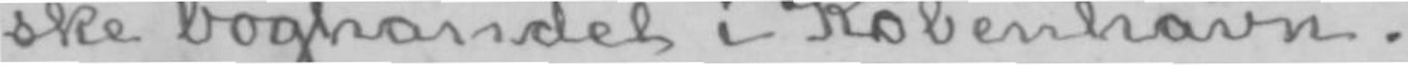ske boghandel i København.
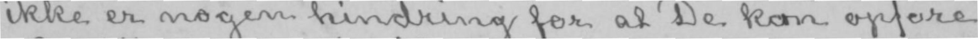ikke er nogen hindring for at De kan opføre
